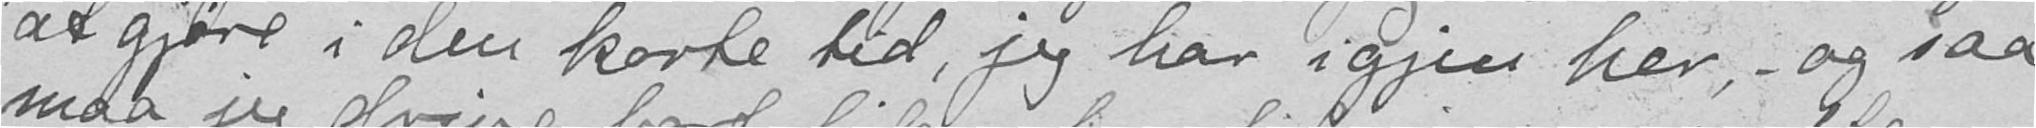at gjøre i den korte tid, jeg har igjen her,- og saa
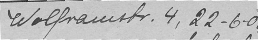Wolframstr. 4, 22-6-01
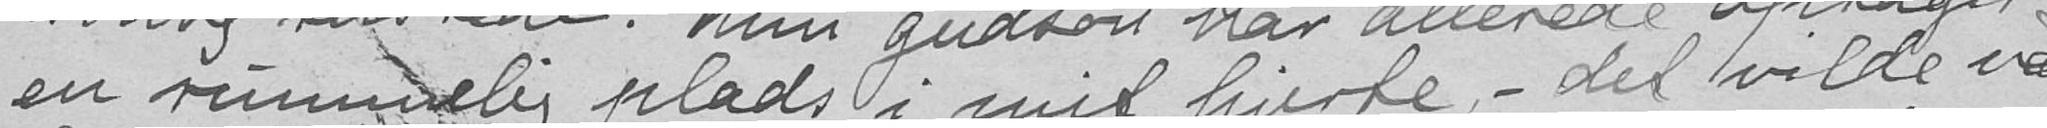en rummelig plads i mit hjerte, - det vilde være
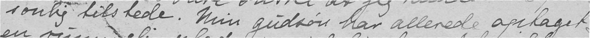sonlig tilstede. Min gudsøn har allerede optaget.
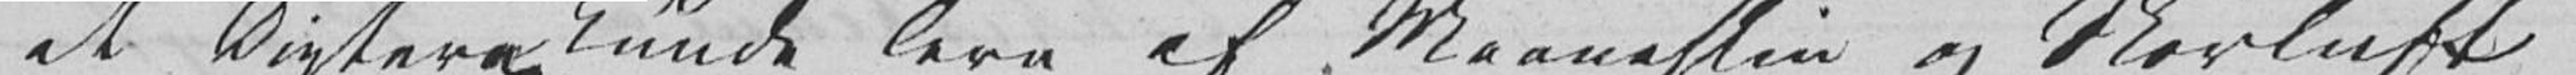at Digter ne kunde leve af Maaneskin og Skovluft
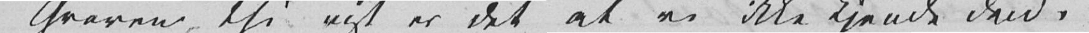Graven, thi vist er det at vi ikke kjende den.
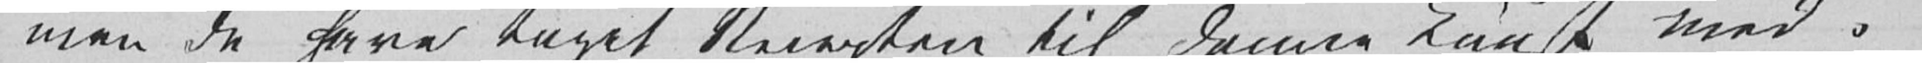men de have taget Recepten til denne Kunst med i
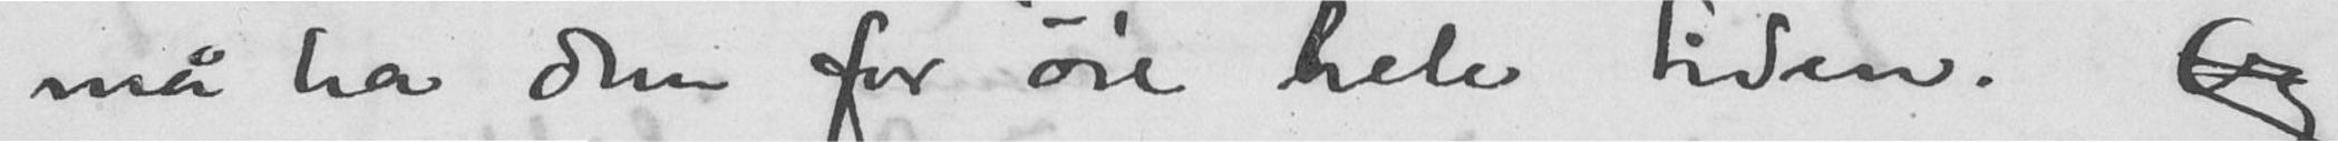må ha dem for øie hele tide. Og
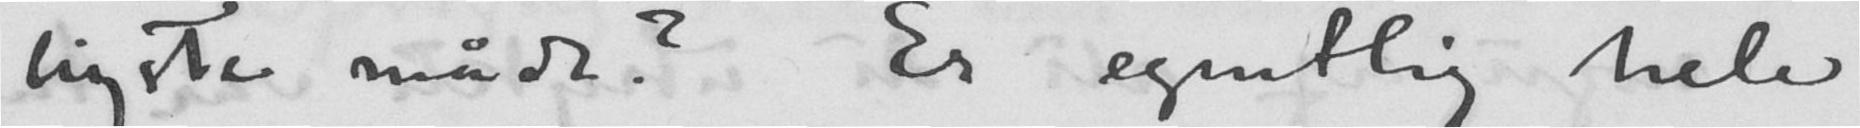ligste måde? Er egentlig hele
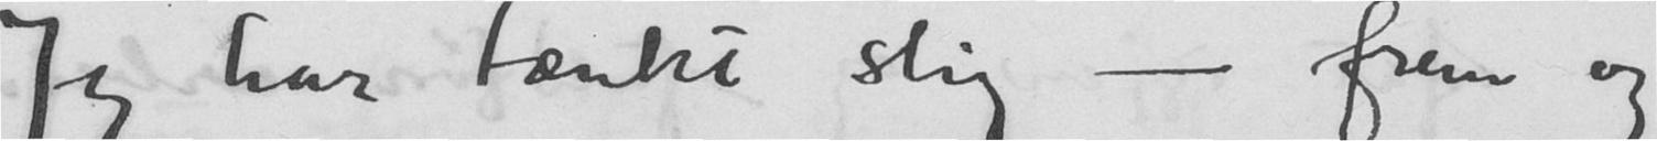Jeg har tænkt slig - frem og
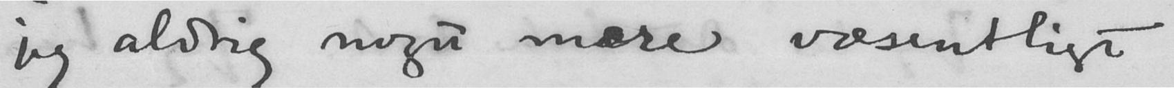jeg aldrig noget mere væsentligt
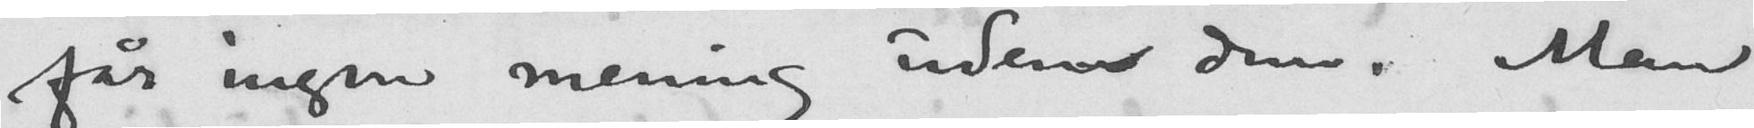får ingen mening uden dem. Man
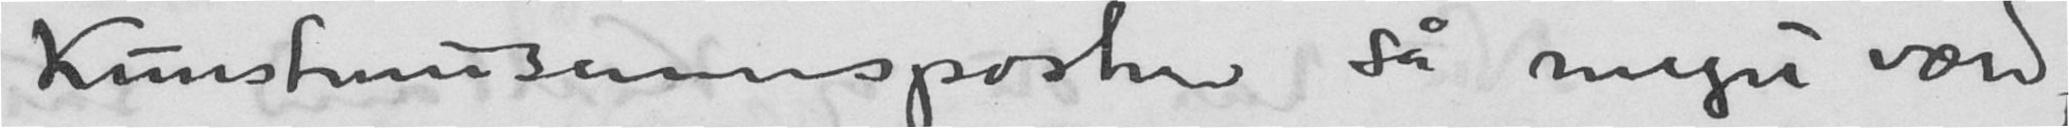kunstmuseumsposten så meget værd,
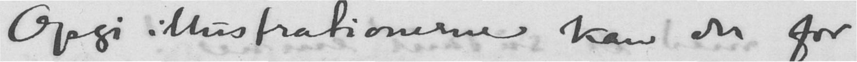Opgi illustrationerne kan der for
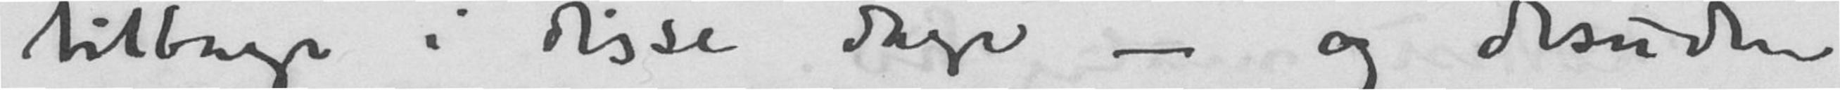tilbage i disse dage - og desuden
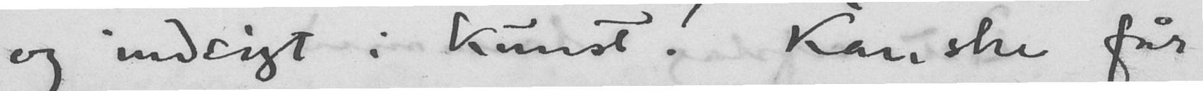og indsigt i kunst! Kanske får
